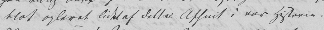blot oplevet lidet af dette Afsnit i vor Historie.
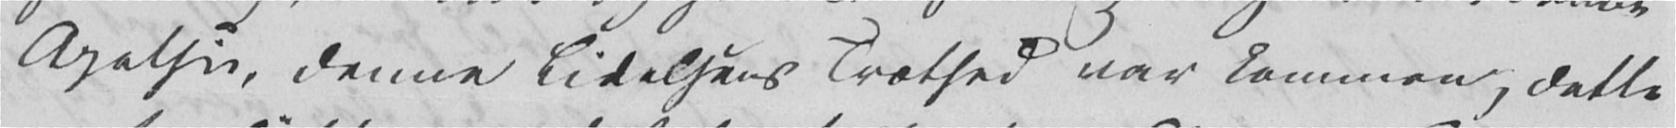Apathie, denne Lidelsens Træthed var kommen, dette
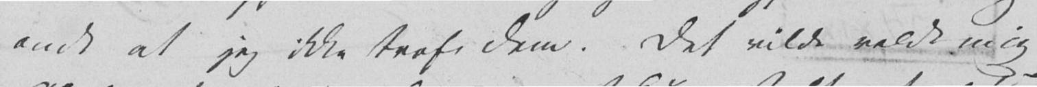ondt at jeg ikke traf Dem. Det vilde voldt mig
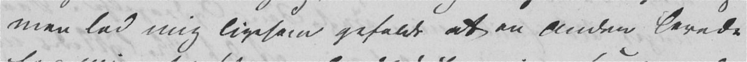men lod mig ligesom gefalde at en Anden levede
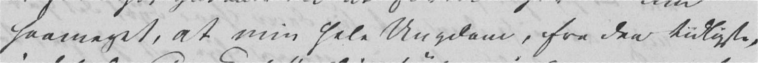saameget, at min hele Ungdom, fra den tidligste,
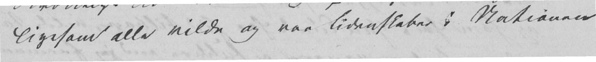ligesom alle vilde og raa Lidenskaber i Nationen
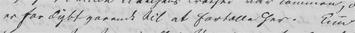er for dybt gaaende til at fortælle her. Kun
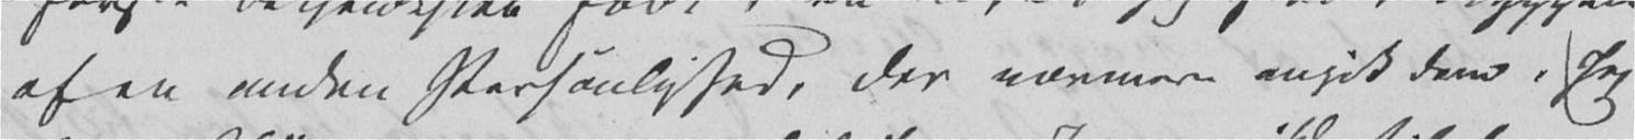af en anden Personlighed, der nærmere angik Dem. Jeg
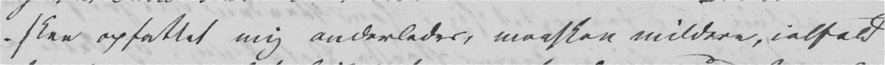skee opfattet mig anderledes, maaskee mildere, ialfald
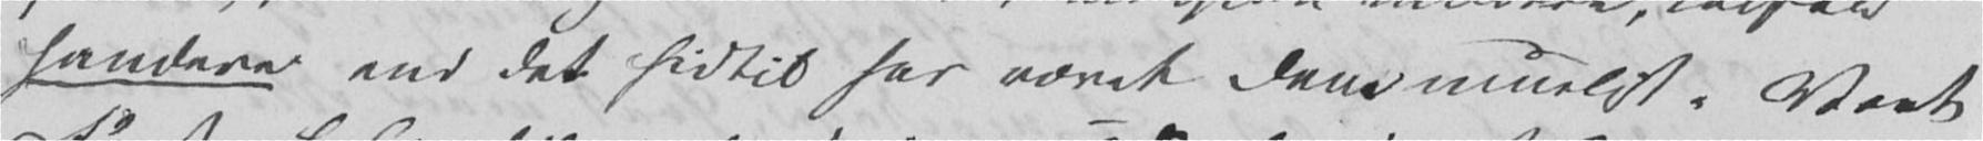sandere end det hidtil har været Dem mueligt. Vort
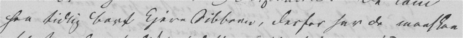saa tidlig bort kjere Sibbern, derfor har De maaskee
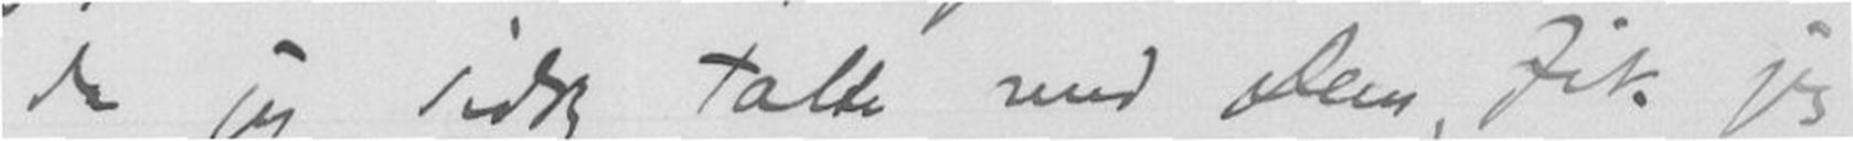da jeg sidst talte med Dem, fik jeg
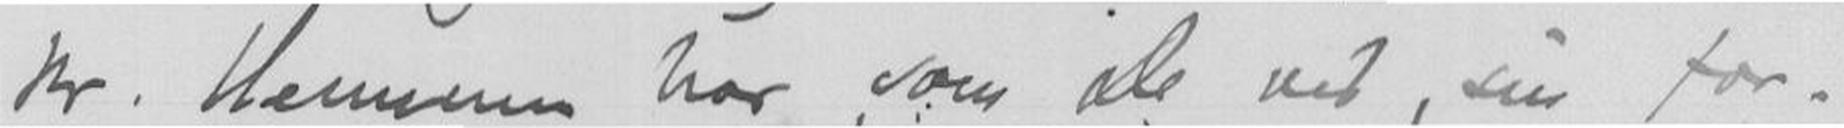Hr. Hennum har som De ved, sin for-
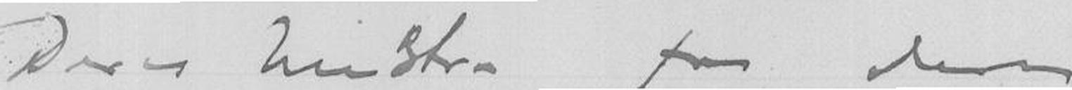Deres hustrufra Deres
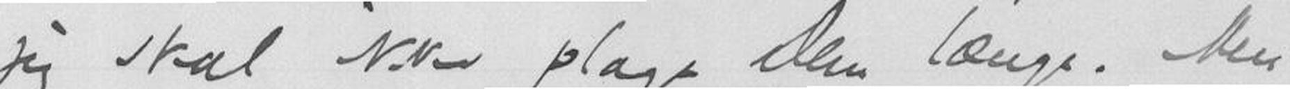jeg skal ikke plage Dem længe. Men
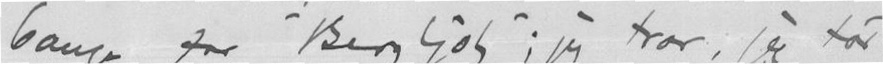bange for "Bergljot"; jeg tror, jeg tør
